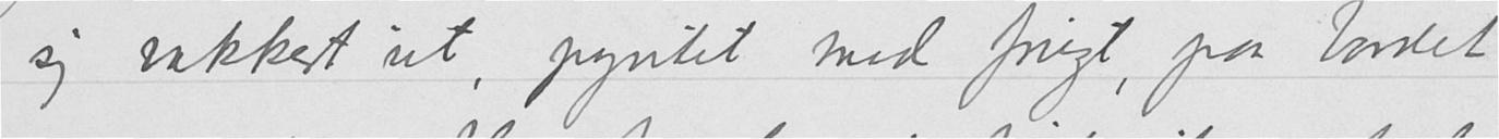sig vakkert ut, pyntet med frugt, paa bordet
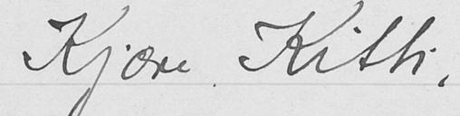Kjære Kitti,
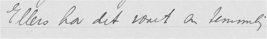Ellers har det været en temmelig
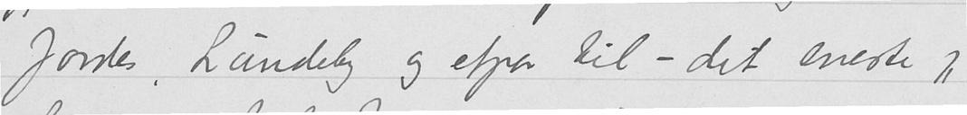Jordes, Lundeby og etpar til – det eneste jeg
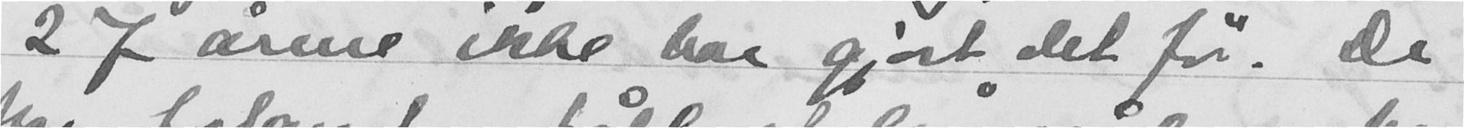27 årene ikke har gjort det før. De
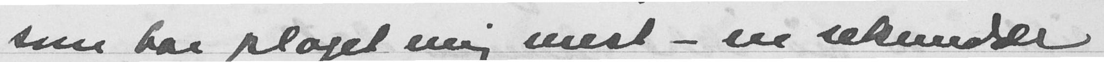som har plaget mig mest - en sekundær
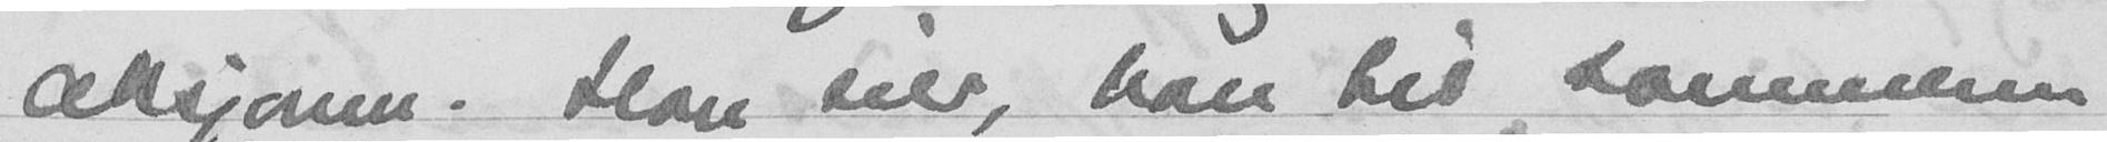aksjonen. Han sier, han til sommeren
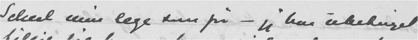Schlel min lege som før - jeg har ubetinget
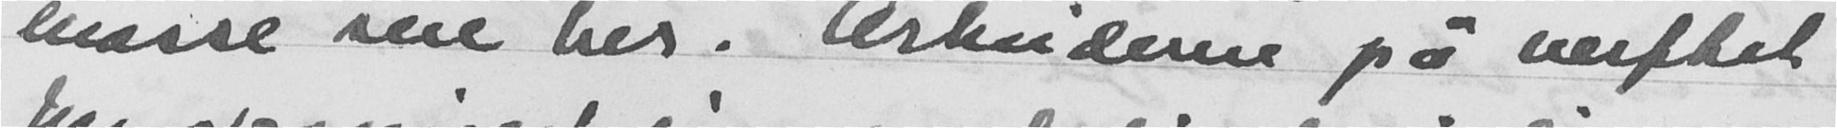masse sne her. Arbeiderne på verftet
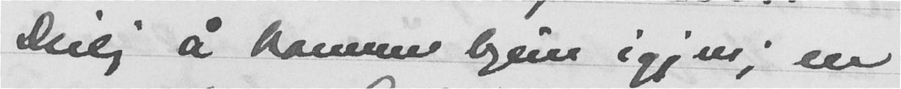Deilig å komme hjem igjen; en
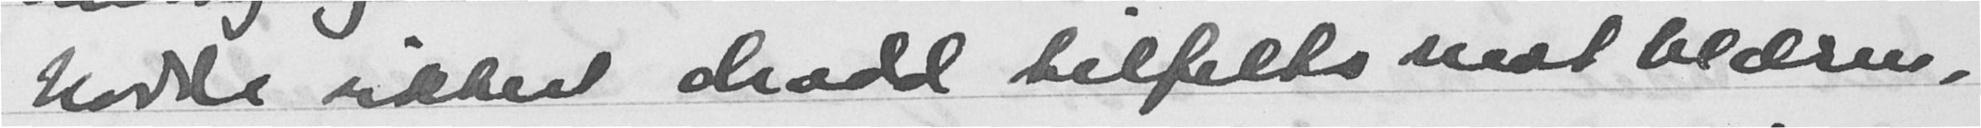hadde sikkert dradd tilfrets mot blæren,
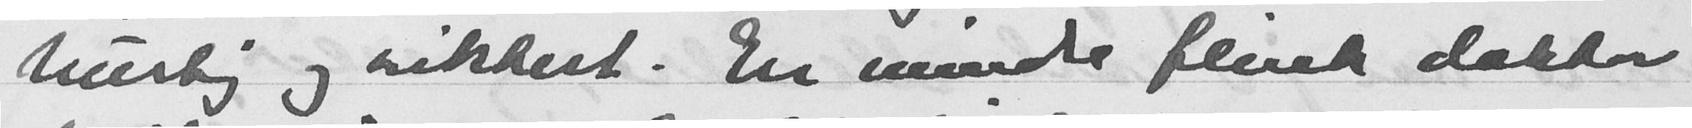hurtig og sikkert. En mindre flink doktor
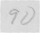90
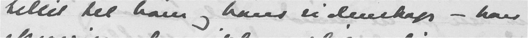tillit til ham og hans videnskap - han
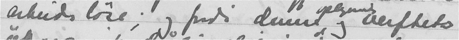arbeidsløse; og fordi denne oplysende og verftets
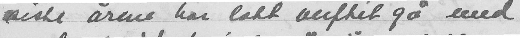siste årene har latt verftet gå med
